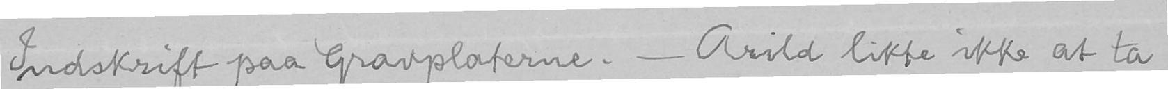Indskrift paa Gravplaterne. - Arild likte ikke at ta
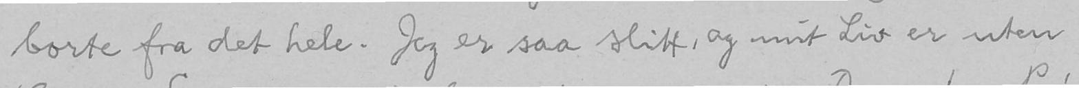borte fra det hele. Jeg er saa slitt, og mit Liv er uten
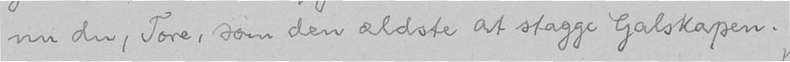nu du, Tore, som den ældste at stagge Galskapen.
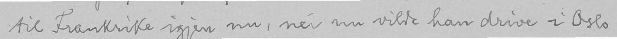til Frankrike igjen nu, nei nu vilde han drive i Oslo
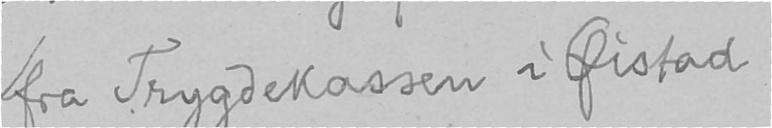fra Trygdekassen i Øistad
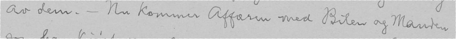av dem. - Nu kommer Affæren med Bilen og Manden
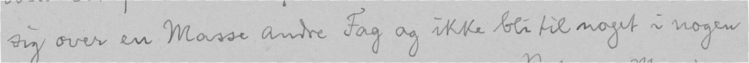sig over en Masse andre Fag og ikke bli til noget i nogen
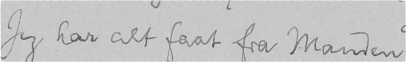Jeg har alt faat fra Manden
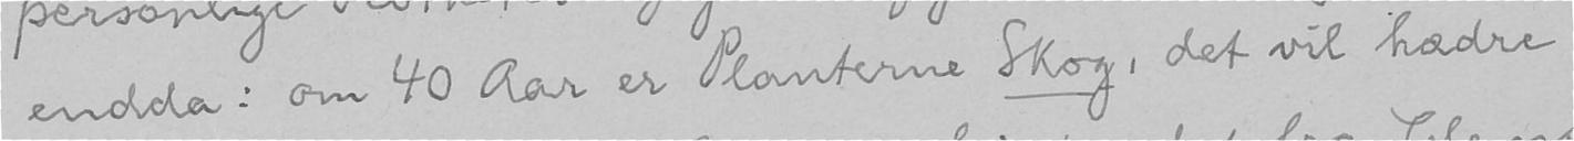endda: om 40 Aar er Planterne Skog, det vil hædre
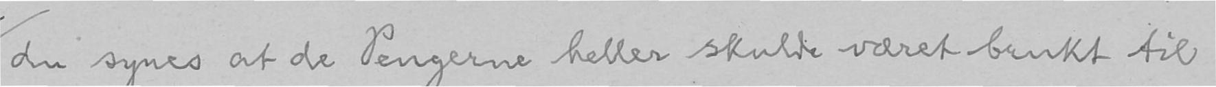du synes at de Pengerne heller skulde været brukt til
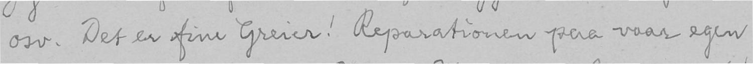osv. Det er fine Greier! Reparationen paa vaar egen
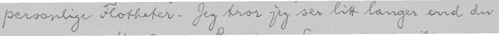personlige Flotheter. Jeg tror jeg ser litt længer end du
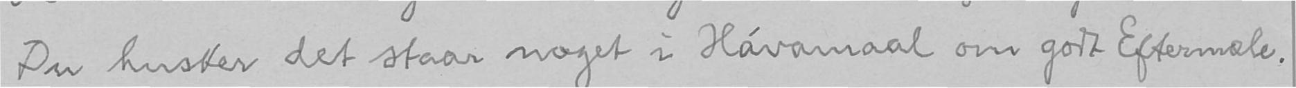Du husker det staar noget i Hávamaal om godt Eftermæle.
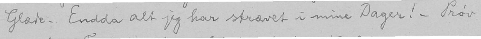Glæde. Endda alt jeg har strævet i mine Dager! - Prøv
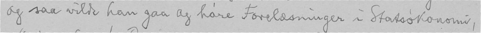og saa vilde han gaa og høre Forelæsninger i Statsøkonomi,
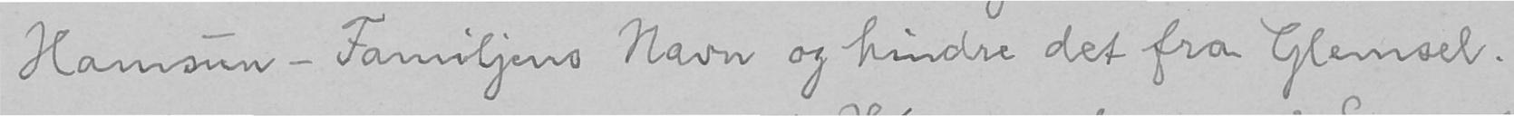Hamsun-Familjens Navn og hindre det fra Glemsel.
